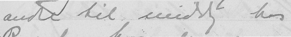andre til middag hos
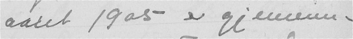aaret 1905 er gjennem-
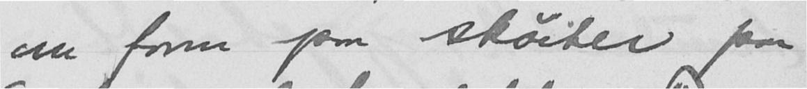om form paa skøiter paa
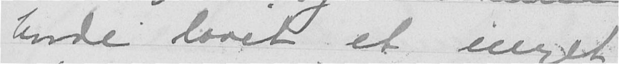havde lavet et meget
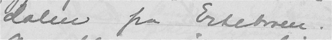dalen fra Ertebroen.
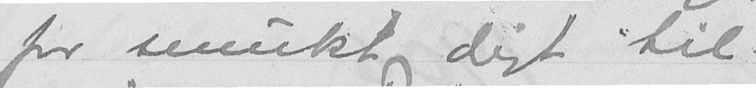for smukt digt til
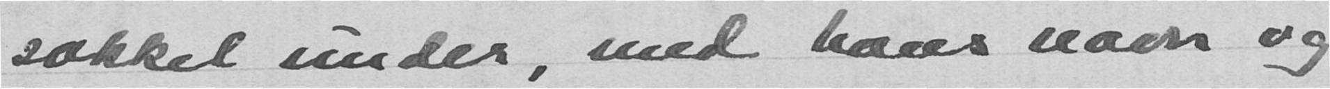sokkel under, med hans navn og
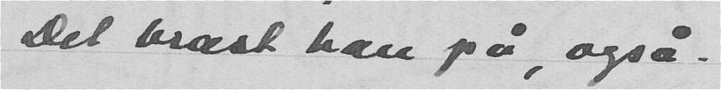Det brast han på, også.
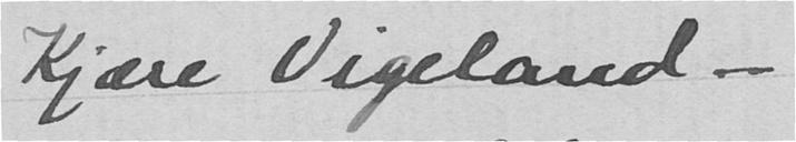Kjære Vigeland
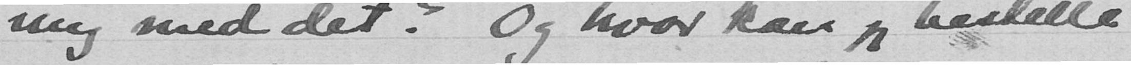mig med det? Og hvor kan jeg bestille
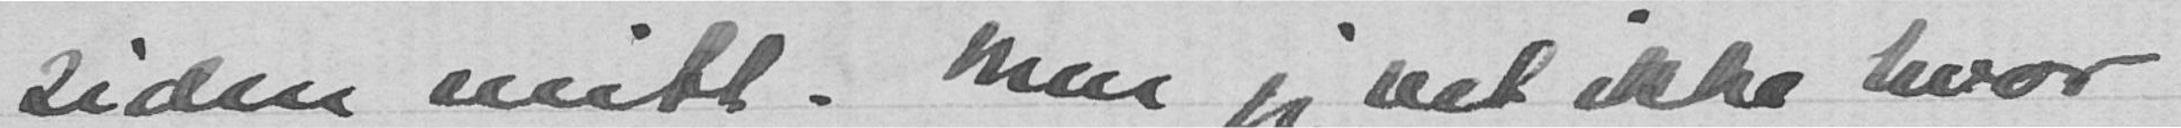siden mitt. Men jeg vet ikke hvor
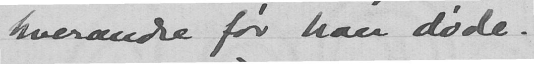hverandre før han døde.
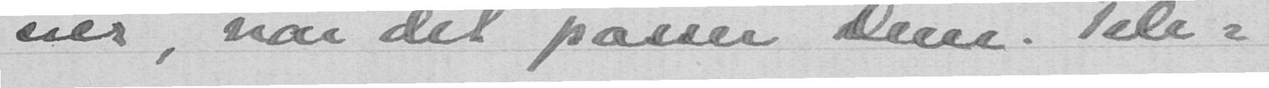sier, når det passer Dem. Tele-
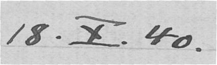18. X. 40.
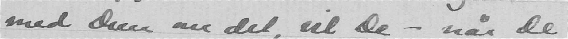med Dem om det, vel De - når De
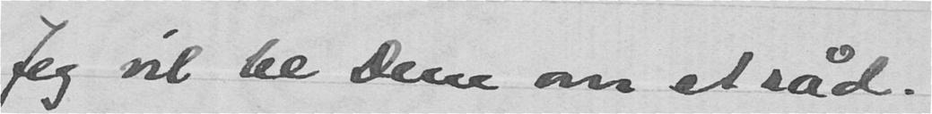Jeg vil be Dem om et råd.
